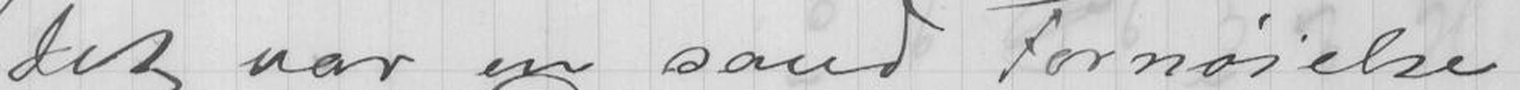Det var en sand Fornøielse
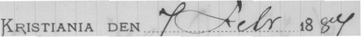Kristiania den 7 Febr 1887.
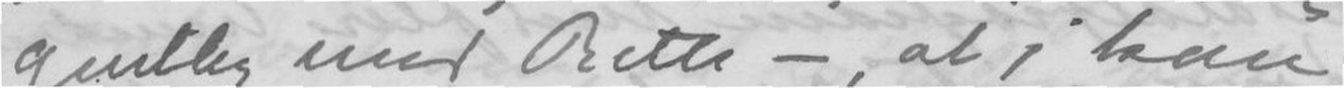gentlig med Rette -, at j kan tage
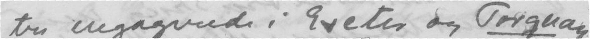tre engagerede i Exctes og Torquay
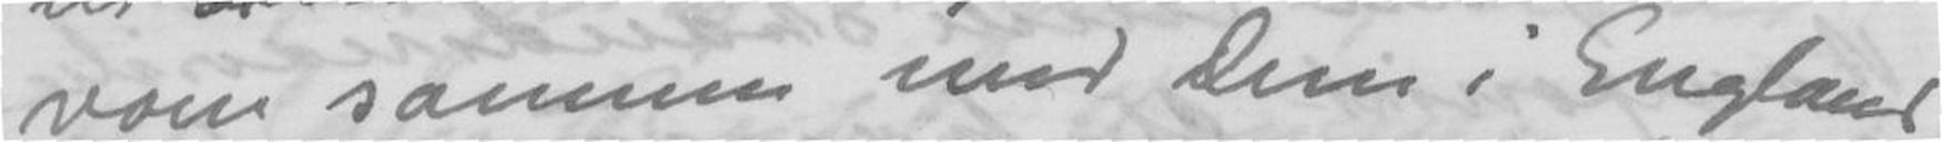vaere sammen med Dem i England
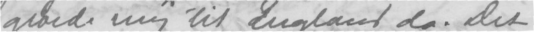glaede mig til England da. Det
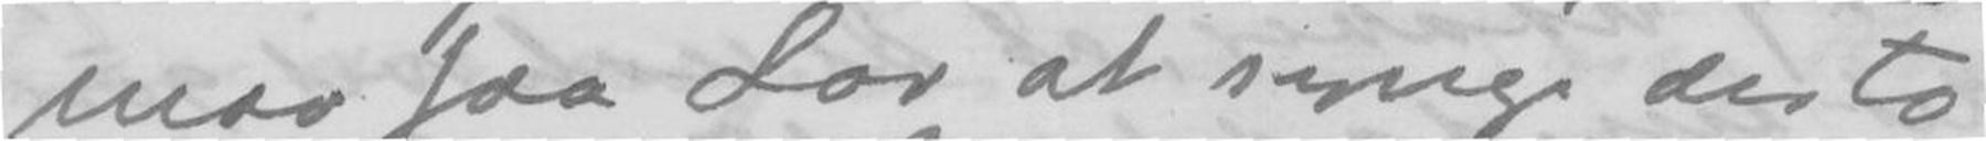maa faa Lov at synge der to
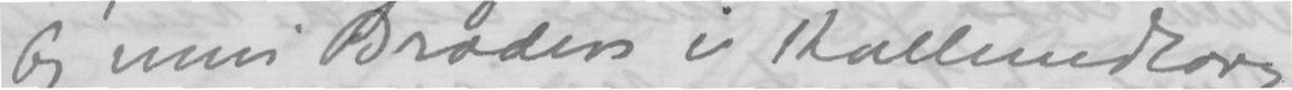Og min Broders i Kallundborg
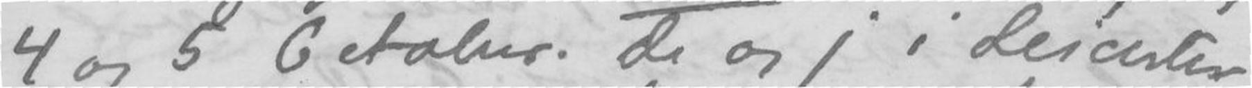4 og 5 October. De og j i Leicester,
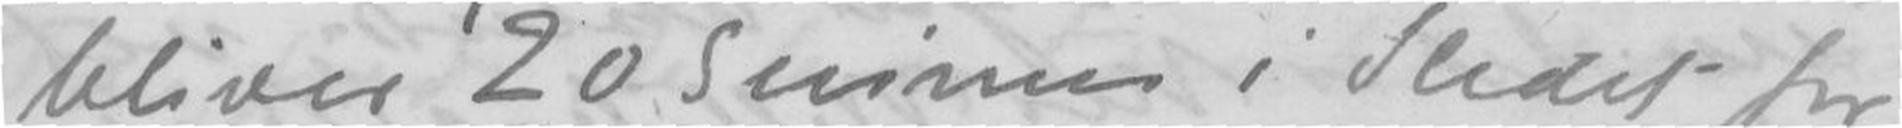bliver 20 Guimer i Stedet for
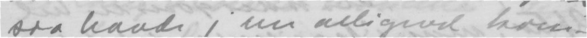saa havde j nu alligevel kom-
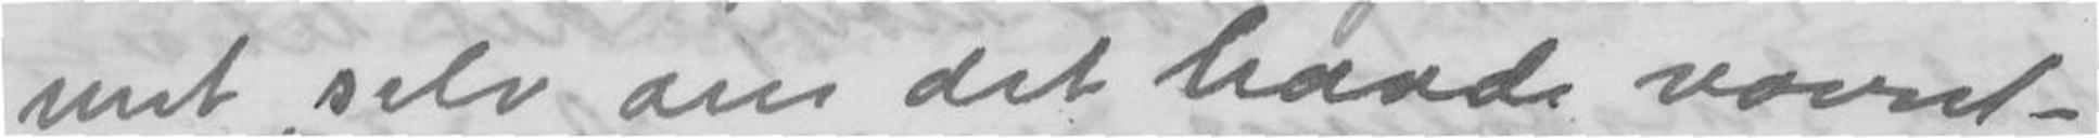met selv om det havde vaeret
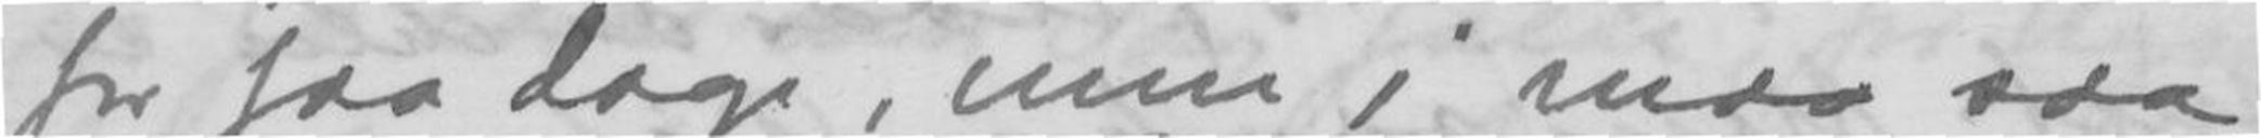for faa Dage, men j maa saa
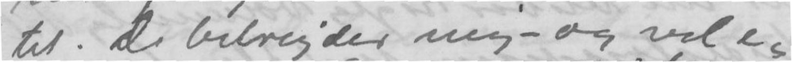til. De bebrejder mig - og vel e-
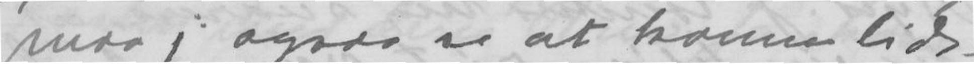maa j ogsaa se at komme lidt
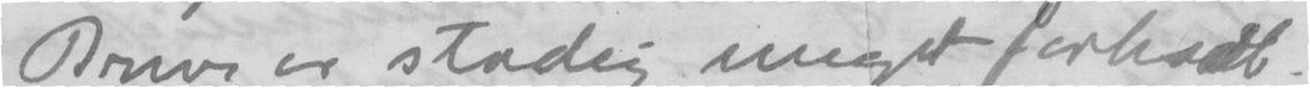Breve er stadig meget forhaab-
